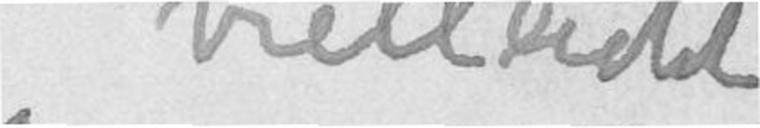vielleicht
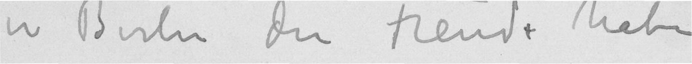in Berlin die Freude haben
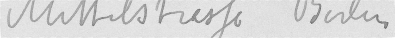Mittelstrasse Berlin
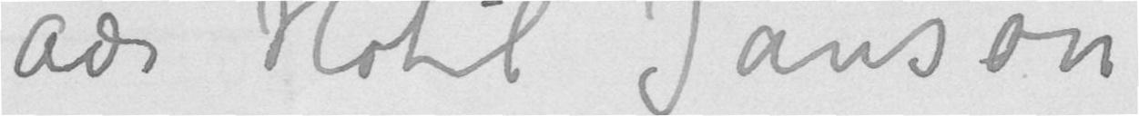Adr Hotel Janson
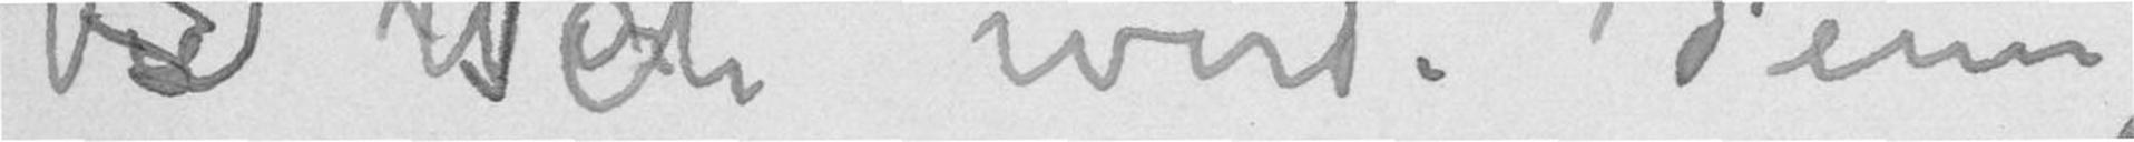Vie Ich werde Ihnen
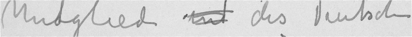Mitglieddes Deutschen
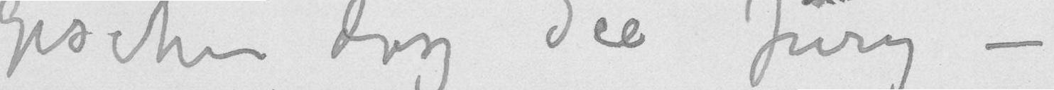gesehen dasz Sie Jury-
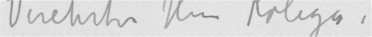Verehrter Herr Kolega!
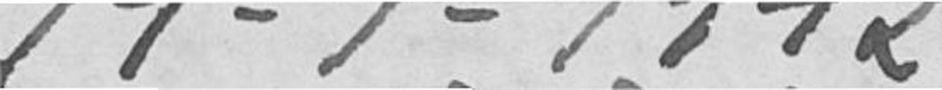19-7-1942
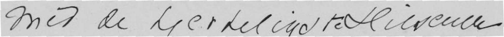Med de hjerteligste Hilsener
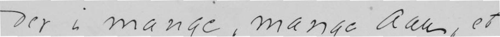der i mange, mange Aar, et
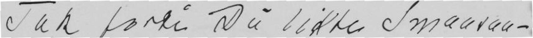Tak fordi Du likte Smaasaa-
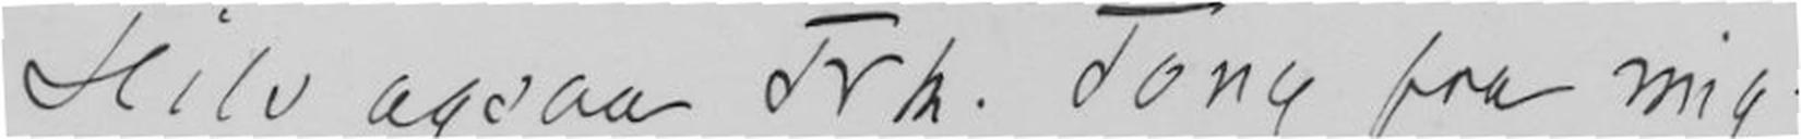Hils ogsaa Frk. Tony fra mig.
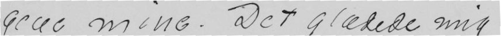gene mine. Det glædede mig -
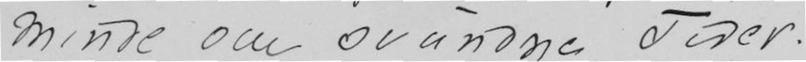minde om svundne Tider.
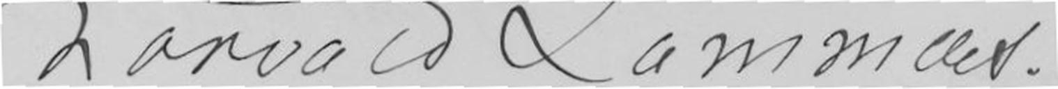Thorvald Lammers
